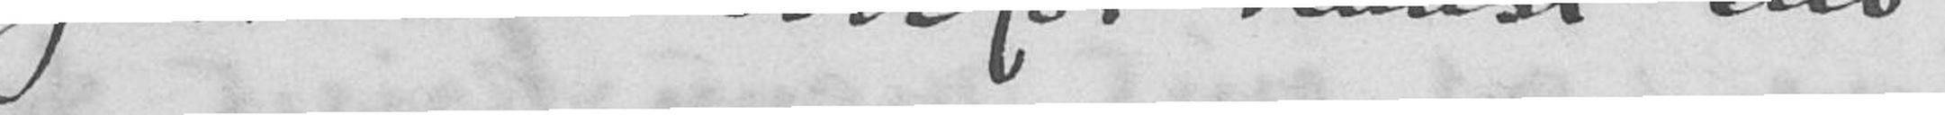judicium overfor kunst end dem
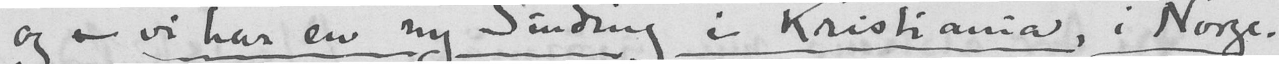og - vi har en ny Sinding i Kristiania, i Norge.
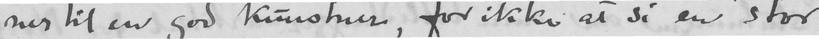ner til en god kunstner, for ikke at si en "stor"
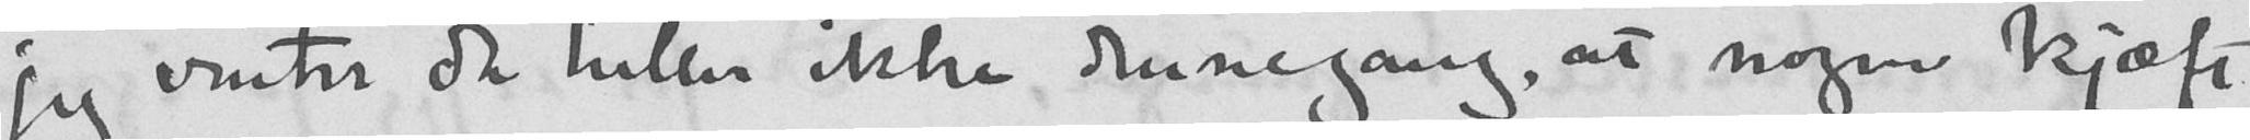jeg venter da heller ikke dennegang, at nogen kjæft
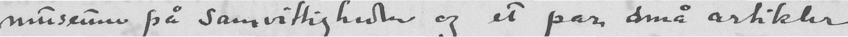museum på samvittigheden og et par små artikler
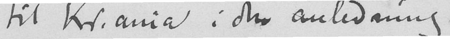til Kr.ania i den anledning. -
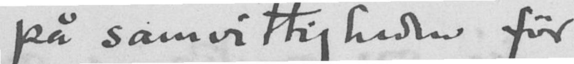på samvittigheden før.
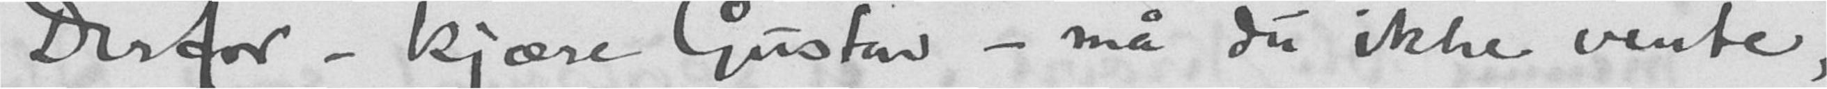Derfor - kjære Gustav - må du ikke vente,
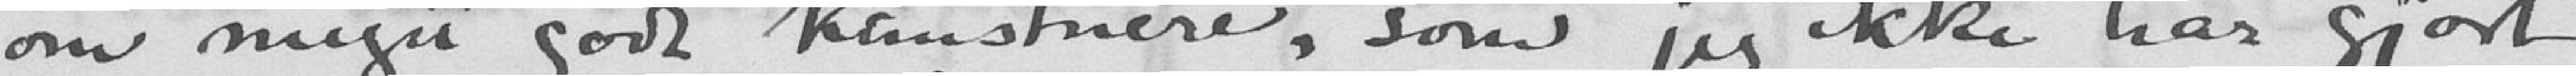om meget gode kunstnere, som jeg ikke har gjort
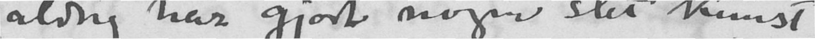aldrig har gjort nogen slet kunst-
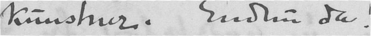kunstner. Endnu da!
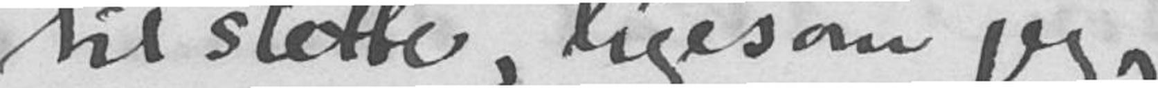til slette, ligesom jeg
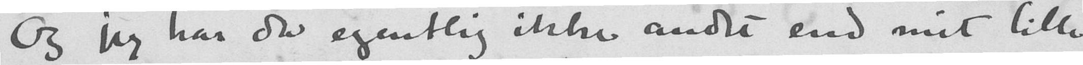Og jeg har da egentlig ikke andet end mit lille
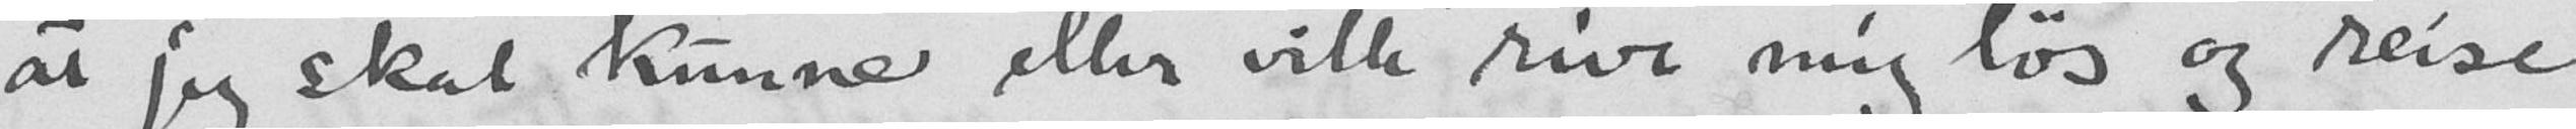at jeg skal kunne eller ville "rive mig løs" og reise
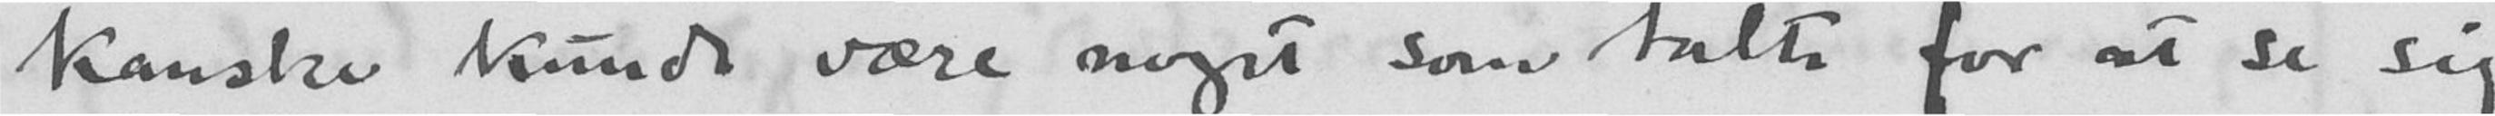kanske kunde være noget som talte for at se sig
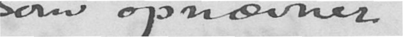som opnævner
